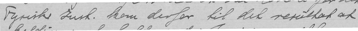Fysiske Inst. kom derfor til det resultat af
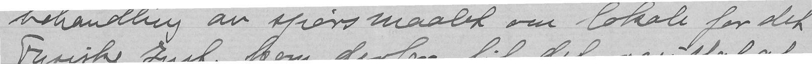behandling av spørsmaalet om lokale for det
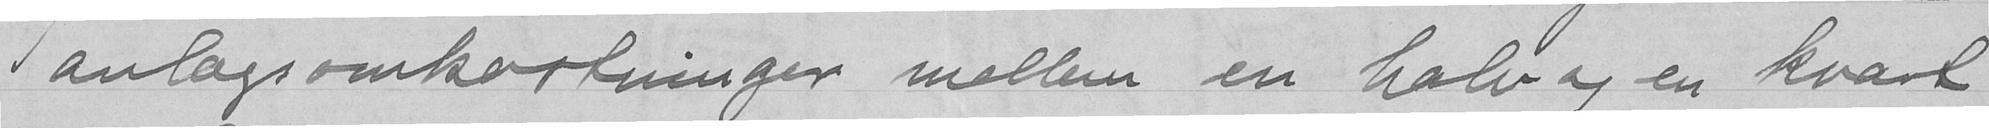anlægsomkostninger mellem en halv og en kvart
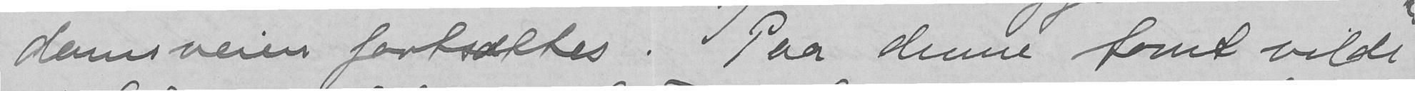damsveien fortsættes.  Paa denne tomt vilde
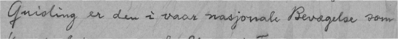Quisling er den i vaar nasjonale Bevægelse som
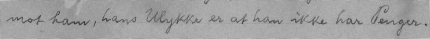mot ham, hans Ulykke er at han ikke har Penger.
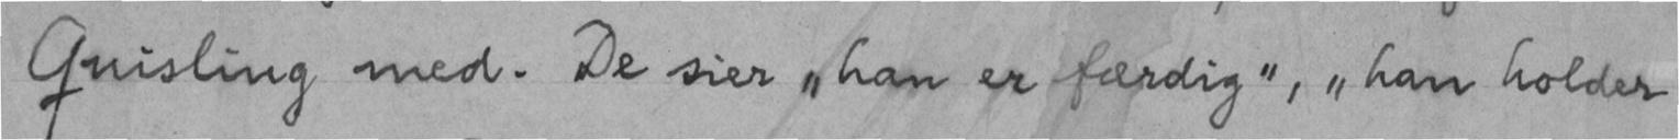Quisling med. De sier "han er færdig", "han holder
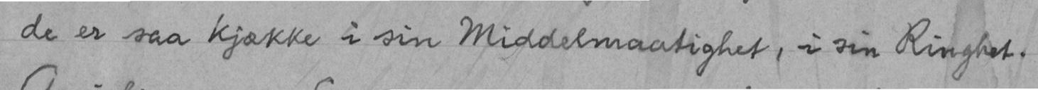de er saa kjække i sin Middelmaatighet, i sin Ringhet.
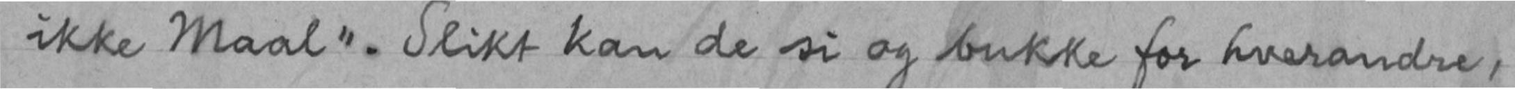ikke Maal". Slikt kan de si og bukke for hverandre,
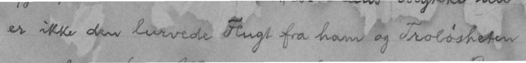er ikke den lurvede Flugt fra ham og Troløsheten
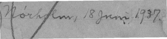Nørholm, 18 Juni 1937.
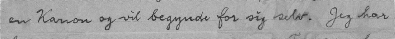en Kanon og vil begynde for sig selv. Jeg har
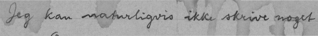Jeg kan naturligvis ikke skrive noget
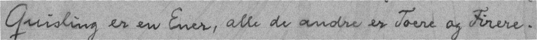Quisling er en Ener, alle de andre er Toere og Firere.
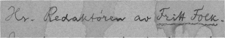Hr. Redaktøren av Fritt Folk.
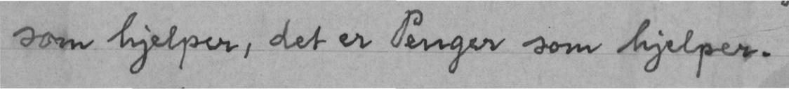som hjelper, det er Penger som hjelper.
